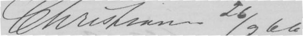Christiania 26/9.66
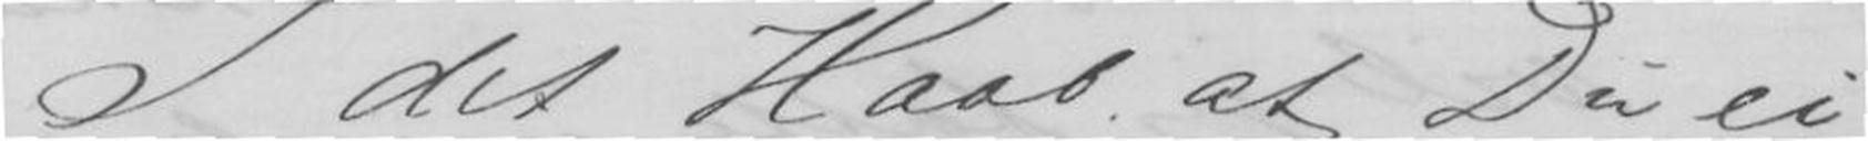I det Haab at Du ei
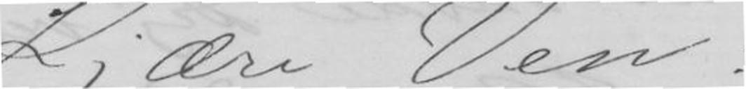Kjære Ven!
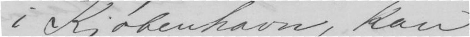i Kjøbenhavn, kan
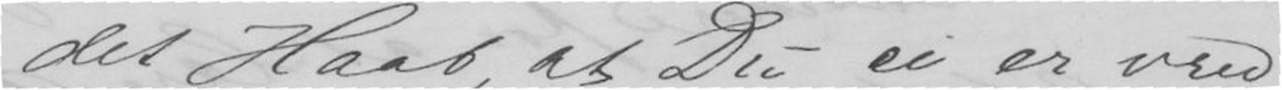det Haab, at Du ei er vred
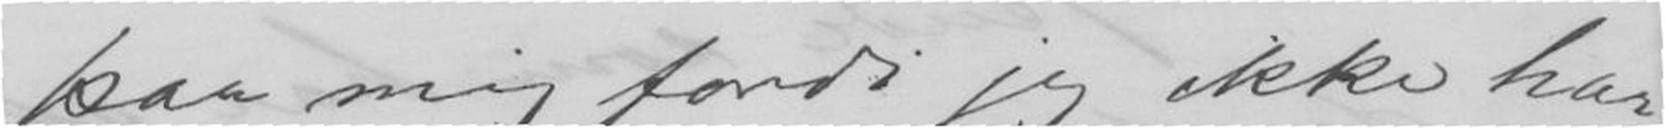paa mig fordi jeg ikke har
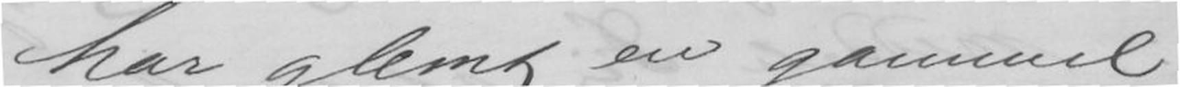har glemt en gammel
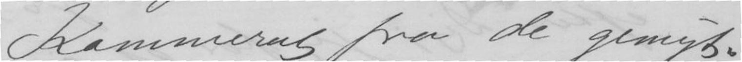Kammerat fra de gemyt-
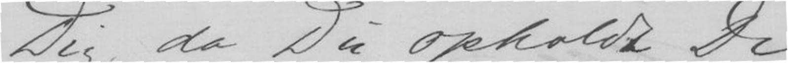Dig da Du opholdt Dig
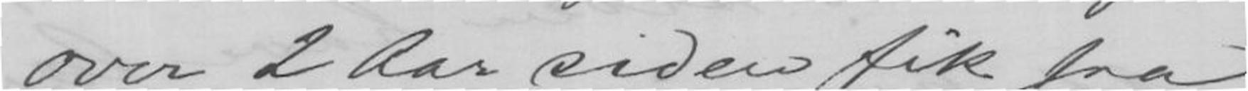over 2 Aar siden fik fra
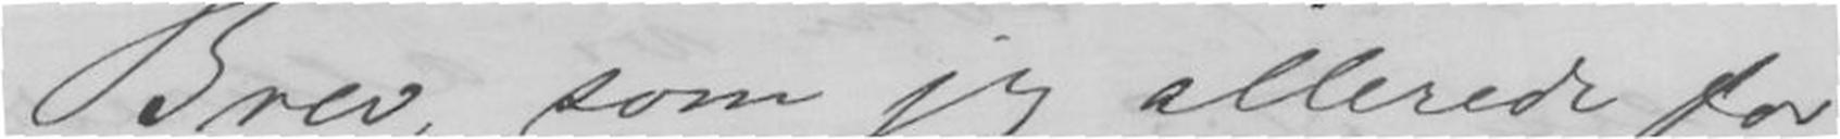Brev, som jeg allerede for
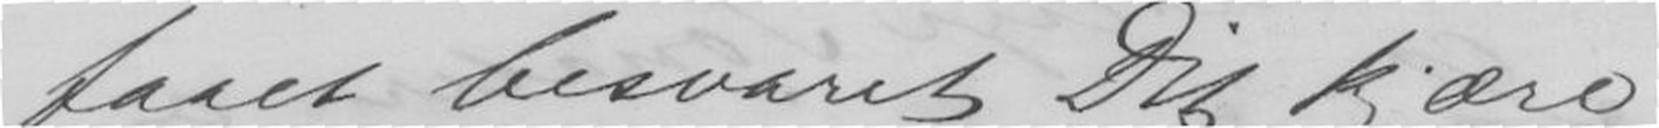faaet besvaret Dit kjære
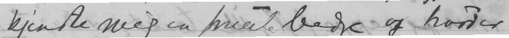kjendte mig en smule bedre og hvad er
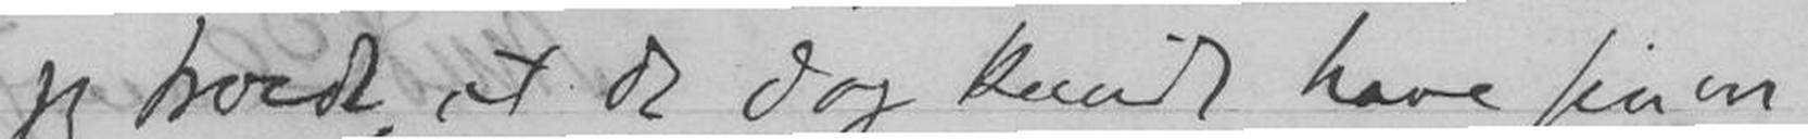jeg troede, at de dog kunde have sin in-
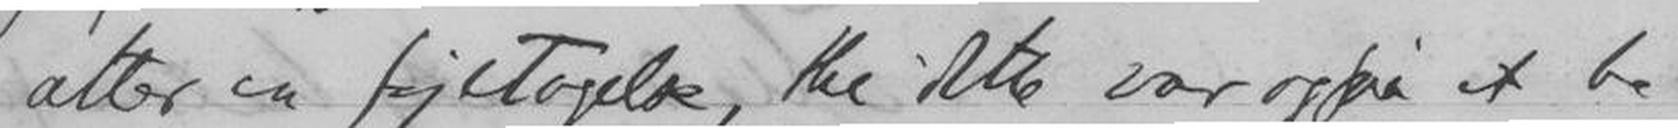atter en fejltagelse, thi dette var også et be-
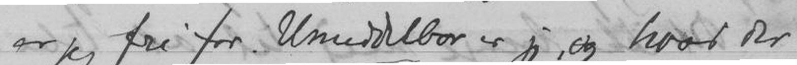er jeg fri for Umiddelbar er jeg, og hvad der
